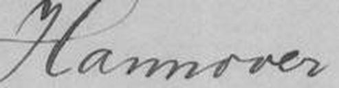Hannover
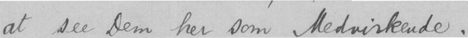at see Dem her som Medvirkende.
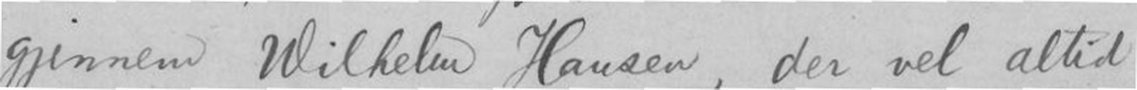gjennem Wilhelm Hausen, der vel altid
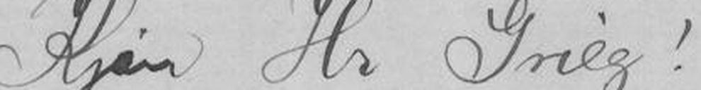Kjære Hr Grieg!
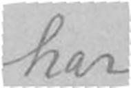har
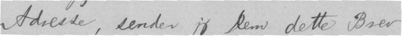Adresse, sender jeg Dem dette Brev
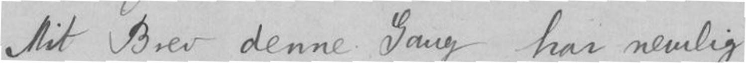Mit Brev denne Gang har nemlig
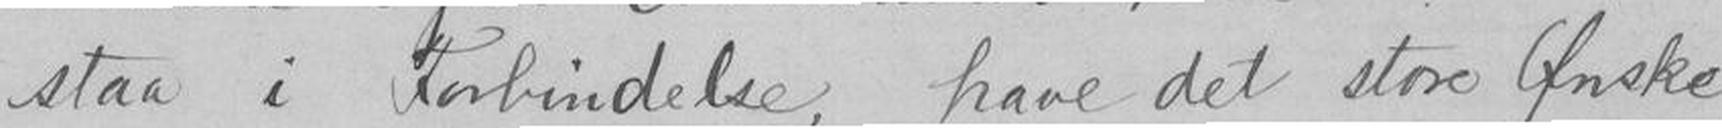staa i Forbindelse, have det store Ønske
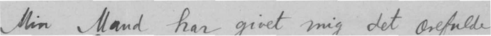Min Mand har givet mig det ærefulde
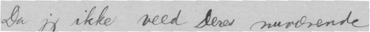Da jeg ikke veed Deres nuværende
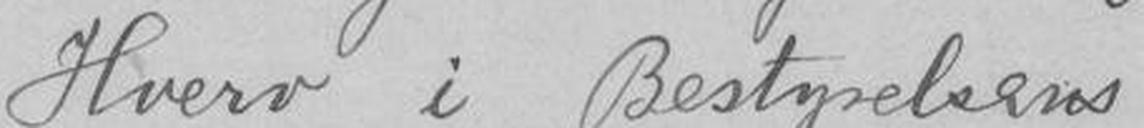Hverv i Bestyrelsens
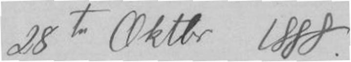28te Oktbr 1888.
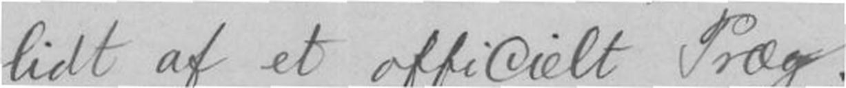lidt af et officielt Præg.
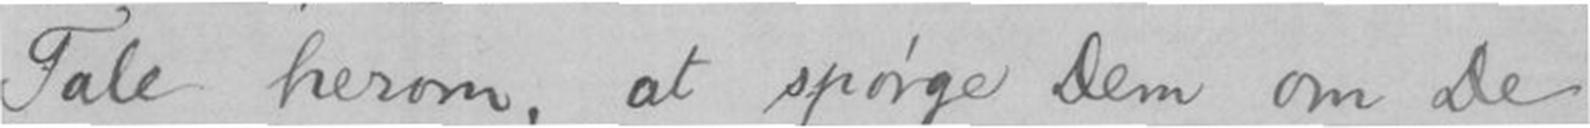Tale herom, at spørge Dem om De
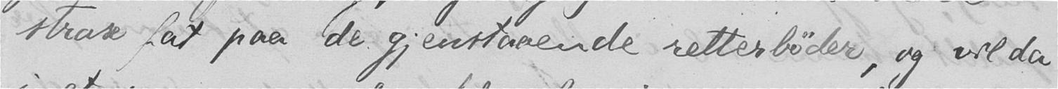strax fat paa de gjenstaaende retterbøder, og vil da
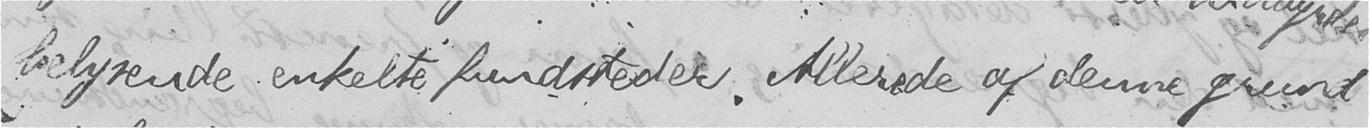belysende enkelte fundsteder. Allerede af denne grund
